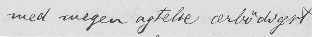med megen agtelse ærbødigst
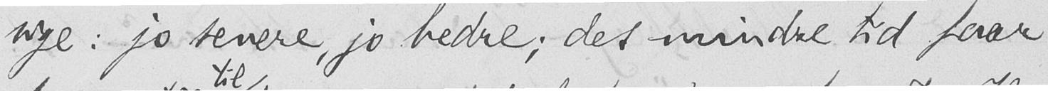sige: jo senere, jo bedre; des mindre tid faar
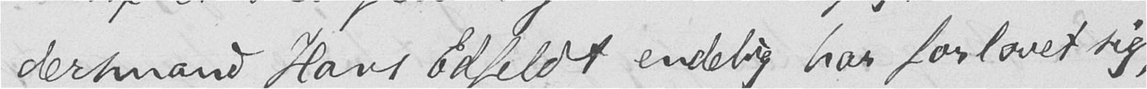dersmand Hans Edfeldt endelig har forlovet sig,
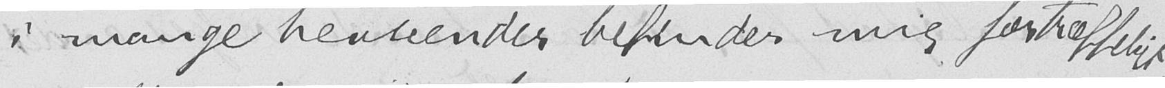i mange henseender befinder mig fortræffeligt.
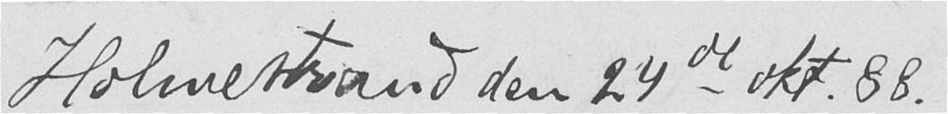Holmestrand den 24de okt. 88.
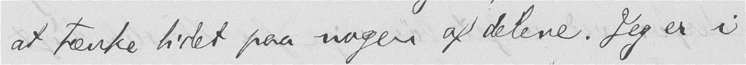at tænke lidet paa nogen af delene. Jeg er i
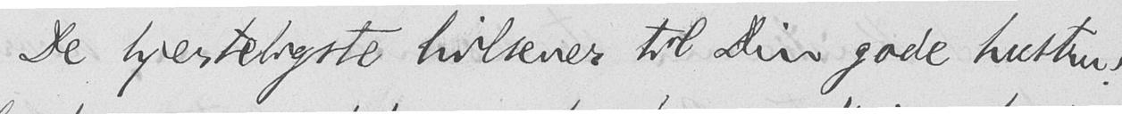De hjerteligste hilsener til Din gode hustru!
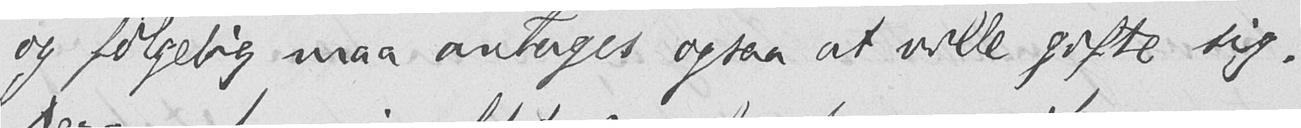og følgelig maa antages ogsaa at ville gifte sig.
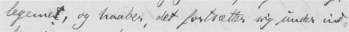legeme, og haaber, det fortsætter sig under ind-
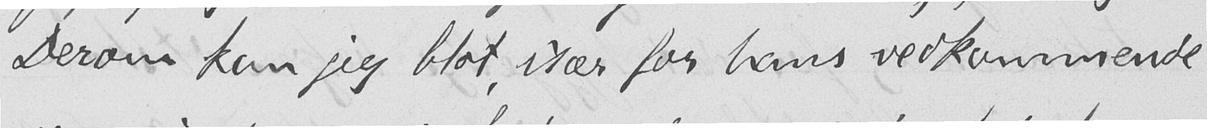Derom kan jeg blot, især for hans vedkommende
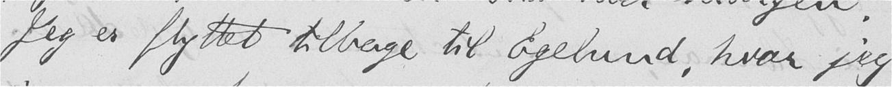Jeg er flyttet tilbage til Egelund, hvor jeg
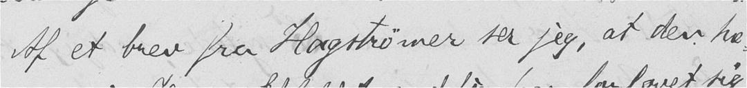Af et brev fra Hagstrømer ser jeg, at den hæ-
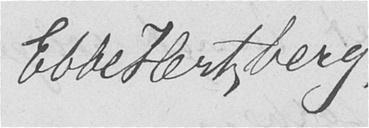Ebbe Hertzberg.
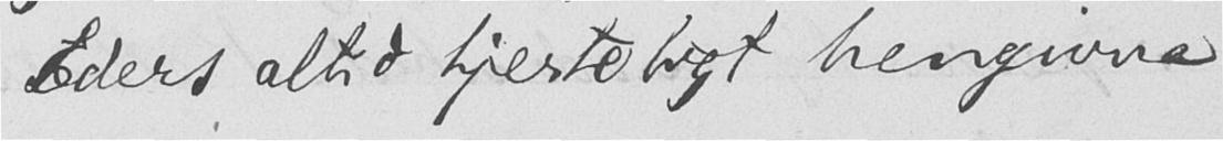Eders altid hjerteligt hengivne
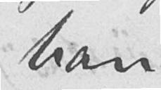han
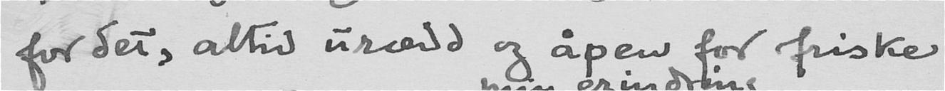for det, altid urædd og åpen for friske
